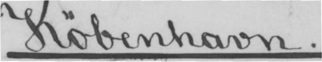København.
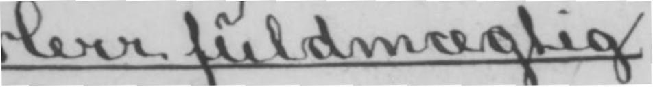Herr fuldmægtig
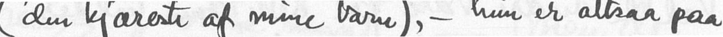(den kjæreste af mine barn), - hun er altsaa paa
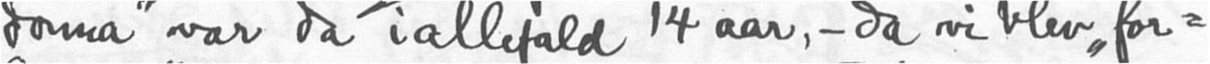donna" var da iallefald 14 aar, - da vi blev "for-
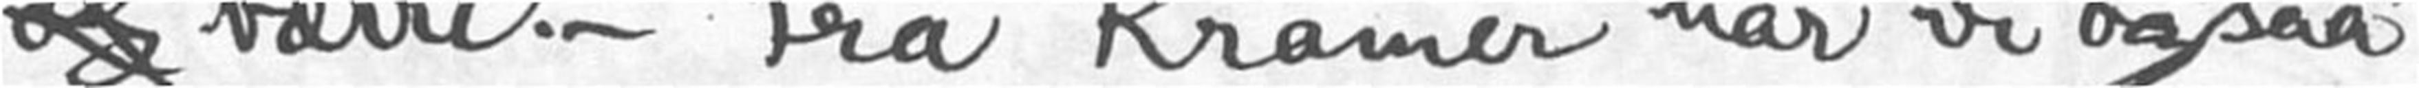værre. - Fra Kramer har vi ogsaa
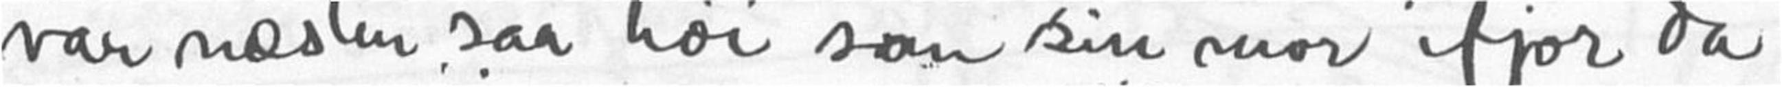var næsten saa høi som sin mor ifjor da
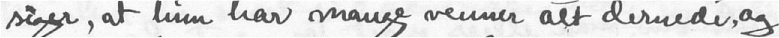siger, at hun har mange venner alt dernede, og
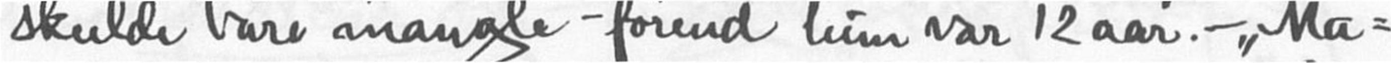skulde bare mangle - førend hun var 12 aar. - "Ma-
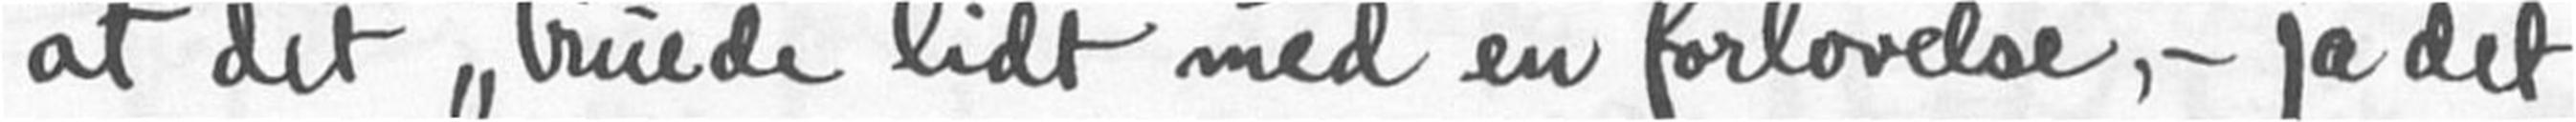at det "truede lidt med en forlovelse", - ja det
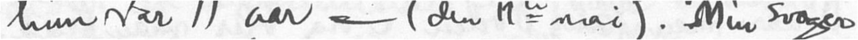hun var 11 aar - (den 11te mai). Min svoger
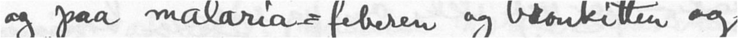og paa malaria-feberen og bronkitten og
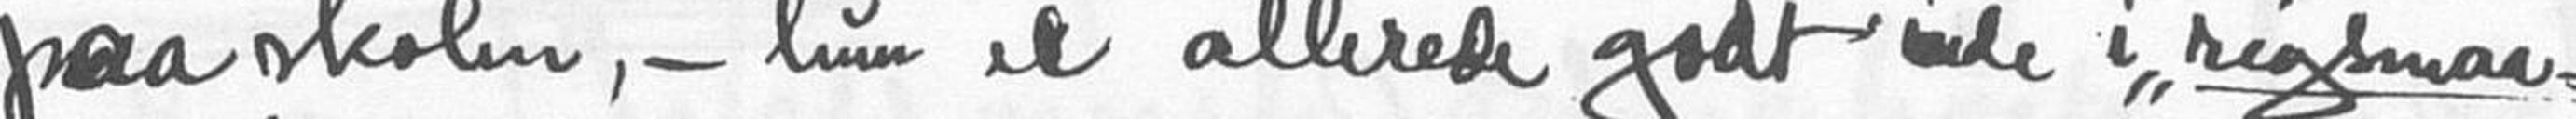paa skolen, - hun er allerde godt inde i "rigsmaa-
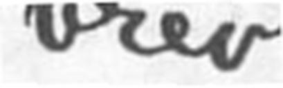brev
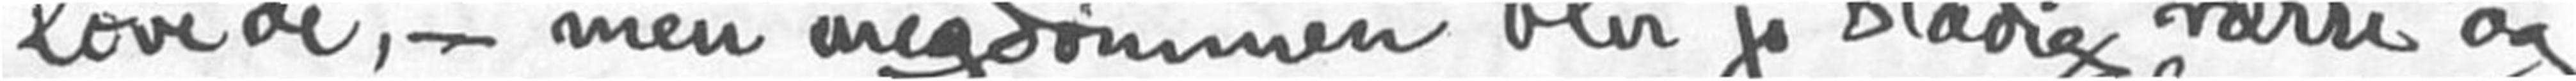lovede", - men ungdommen blir jo stadig værre og
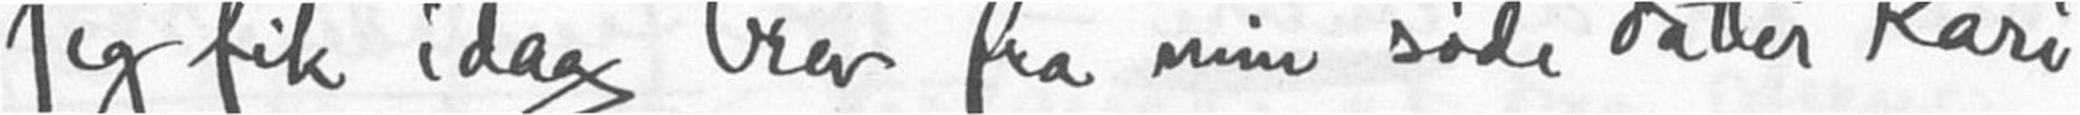Jeg fik idag brev fra min søde datter Kari
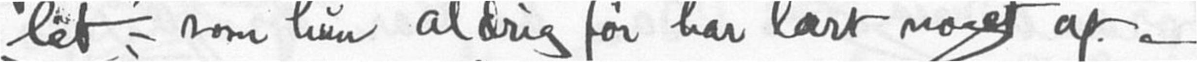let" som hun aldrig før har lært noget af.
